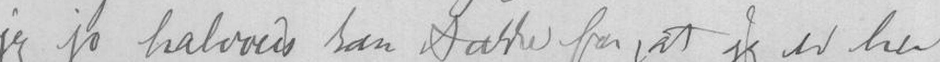jeg jo halveies kan takke for, at jeg er her
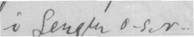i sengen o-s-v.
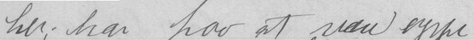her; her Lov at være oppe
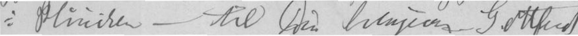i kliniken - til Din hengivne Gottfred M=H.
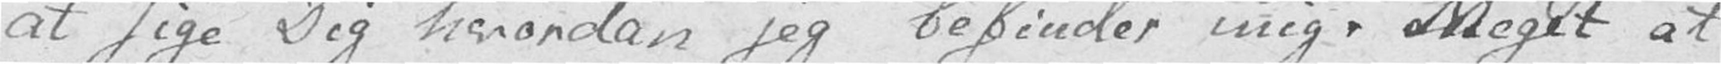at sige Dig hvordan jeg befinder mig. Meget at
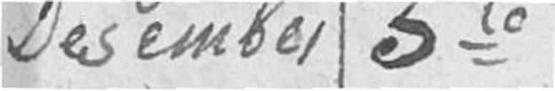Desember 5te
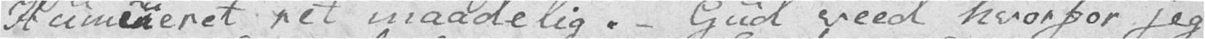Humeueret ret maadelig. – Gud veed hvorfor jeg
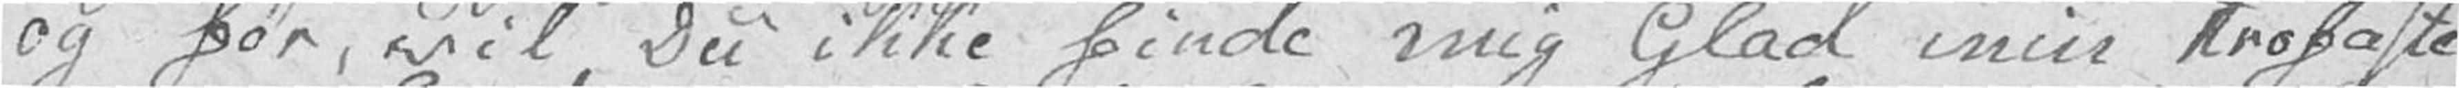og før, vil Du ikke finde mig Glad min trofaste
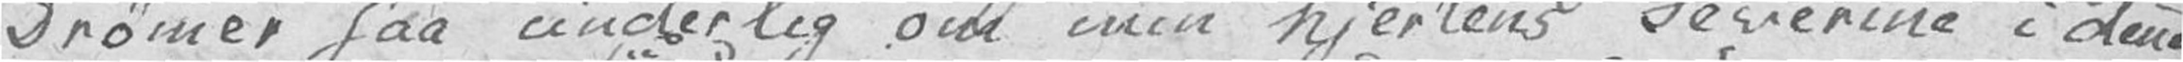Drømmer saa underlig om min Hjertens Severine i denne
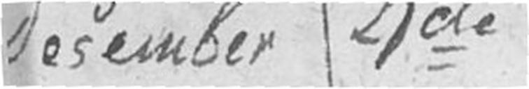Desember 4de
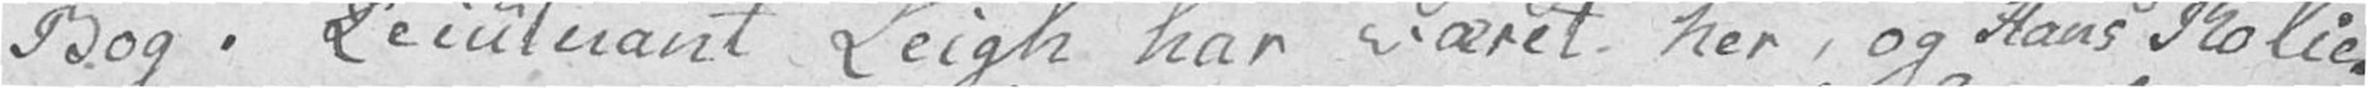Bog. Leiutnant Leigh har været her, og Hans Rolie.
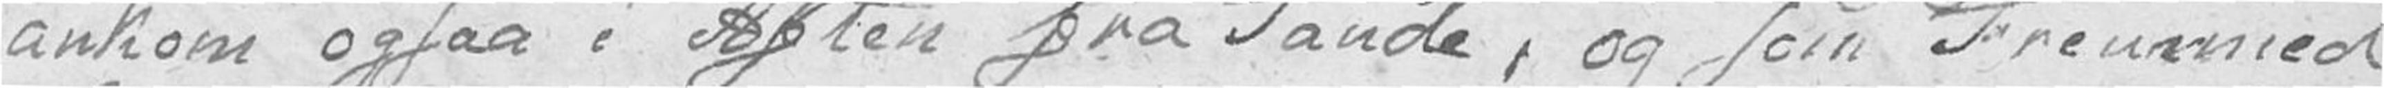ankom ogsaa i Aften fra Tande, og som Fremmed
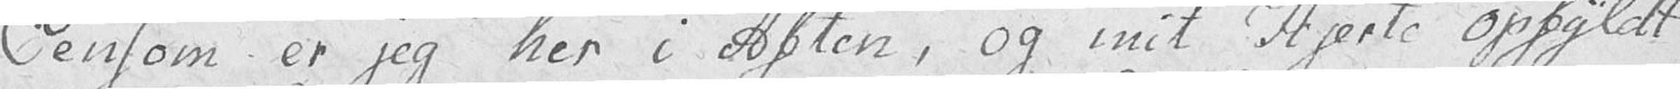Eensom er jeg her i Aften, og mit Hjerte opfyldt
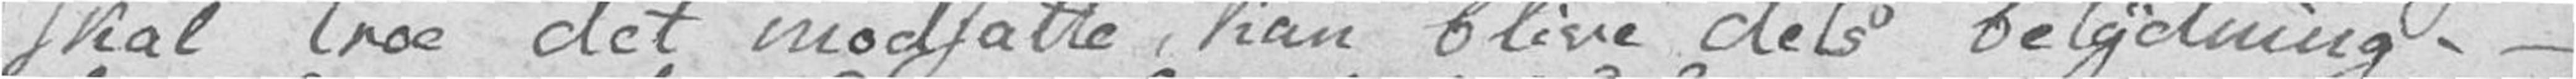skal troe det modsatte, kan blive dets betydning. –
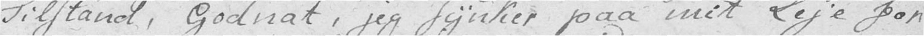Tilstand, Godnat, jeg synker paa mit Leje for
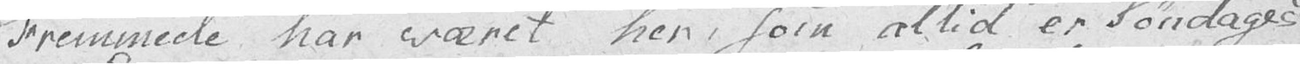Fremmede har været her, som altid er Søndager
